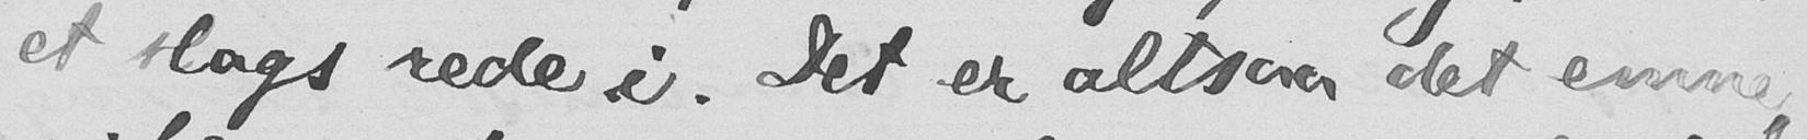et slags rede i. Det er altsaa det emne,
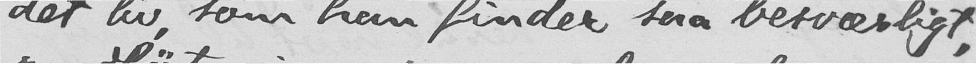det liv, som han finder saa besværligt,
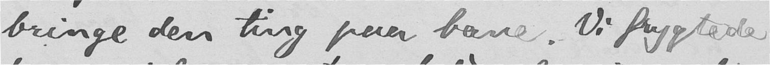bringe den ting paa bane. Vi frygtede
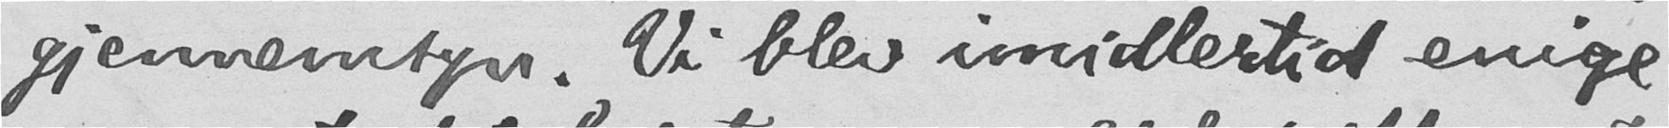gjennemsyn. Vi blev imidlertid enige
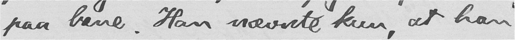paa bane. Han nævnte kun at han
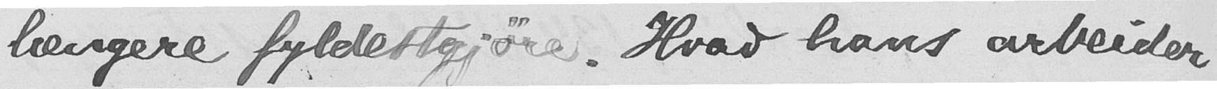længere fyldestgjøre. Hvad hans arbeider
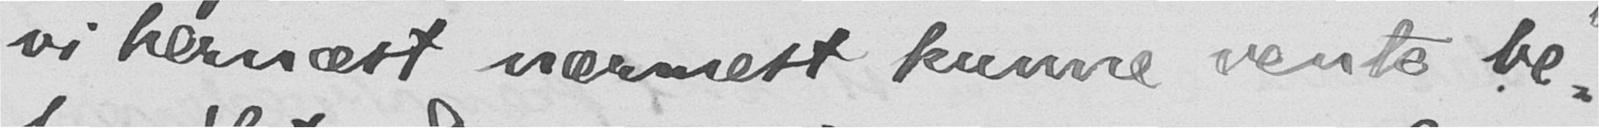vi hernæst nærmest kunne vente be-
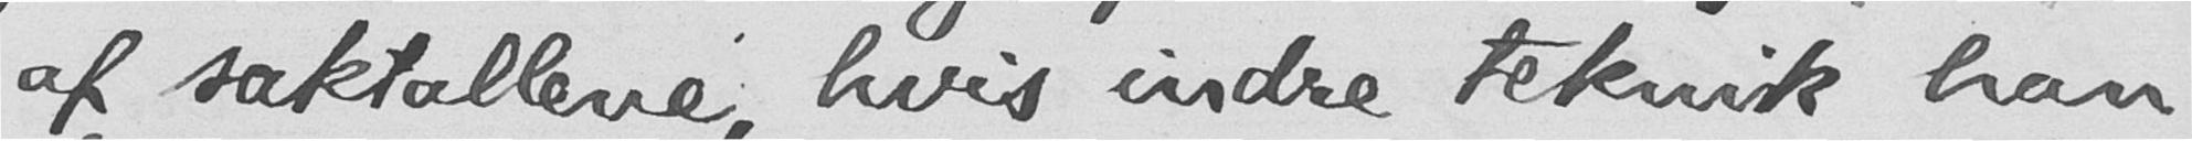af saktallene, hvis indre teknik han
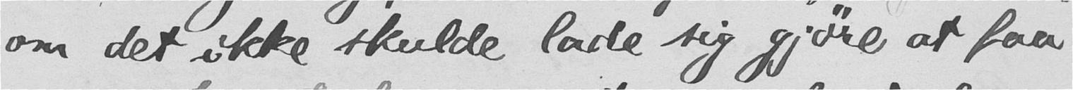om det ikke skulde lade sig gjøre at faa
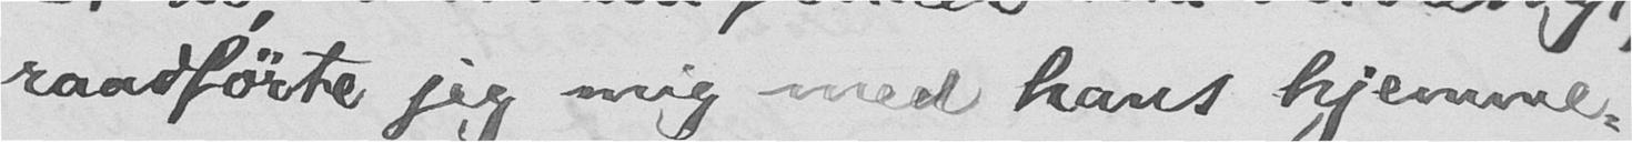raadførte jeg mig med hans hjemme-
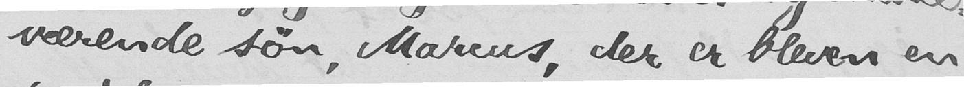værende søn, Marcus, der er bleven en
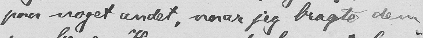paa noget andet, naar jeg bragte dem
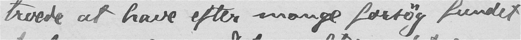troede at have efter mange forsøg fundet
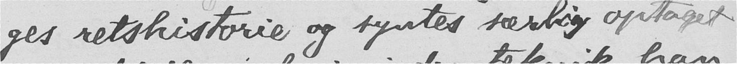ges retshistorie og syntes særlig optaget
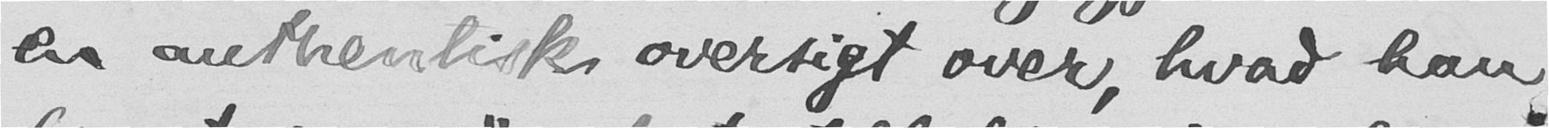en authentisk oversigt over, hvad han
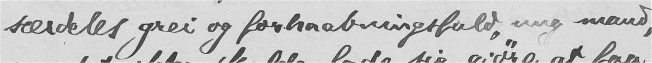særdeles grei og forhaabningsfuld, ung mand,
